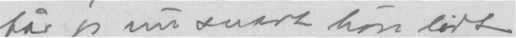får jeg nu snar høre lidt
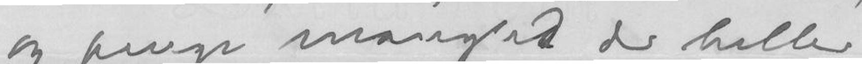og penge manglede de heller
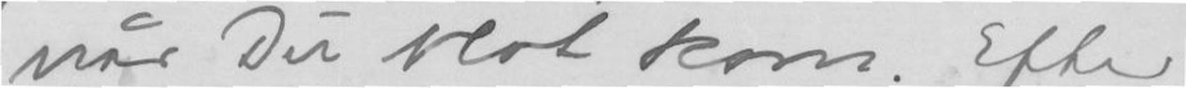når Du blot kom. Efter
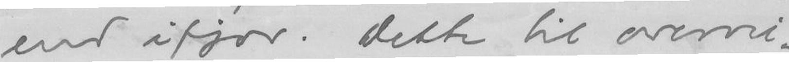end ifjor. Dette til overvei-
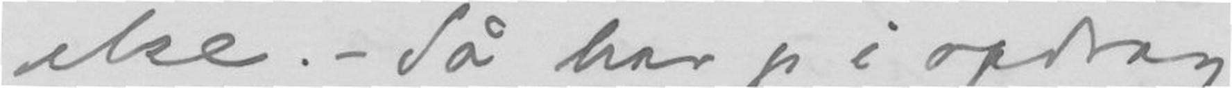else.- Sa har jeg i opdrag
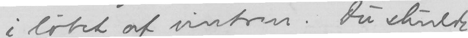i løbet af vinteren. Du skulde
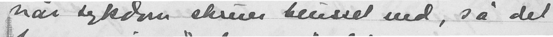når sykdom skrur blusset ned, så det
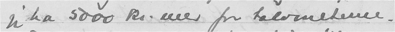jeg ha 5000 kr. mer for tolvmeterne!
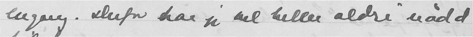engang. Derfor har jeg vel heller aldri nådd
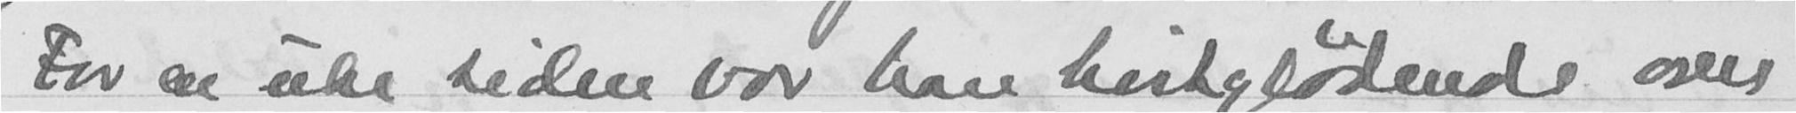For en uke siden var han hvitglødende over
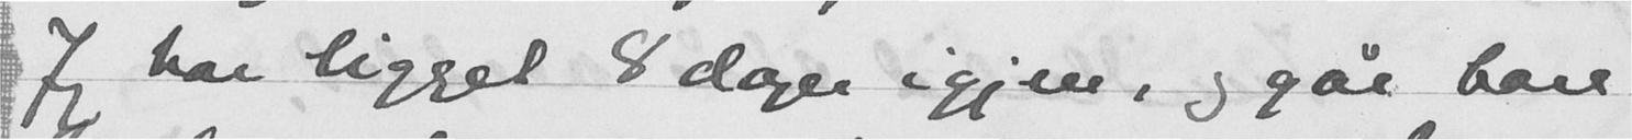Jeg har ligget 8 dager igjen, og går bare
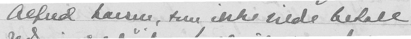Alfred Larsen, som ikke vilde betale
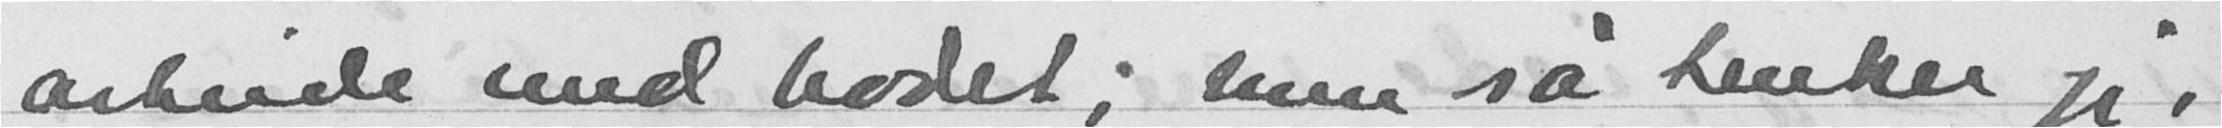arbeide med hodet; men så tenker jeg,
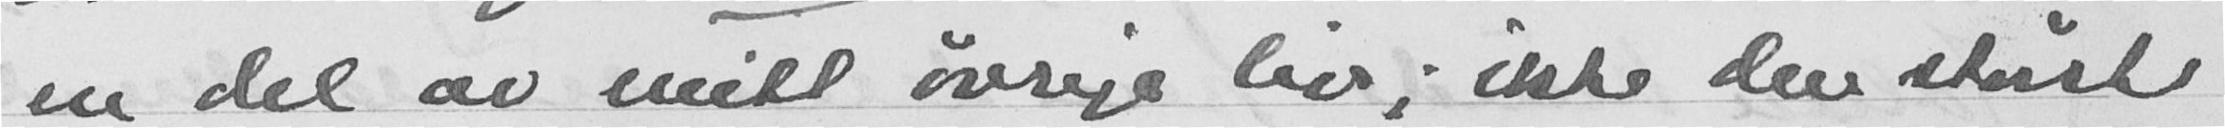en del av midt øvrige liv; ikke den største
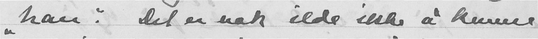"han." Det er nok ilde ikke å kunne
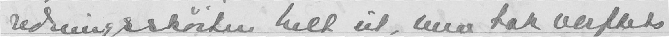redningsskøiten helt ut, men tok verftets
